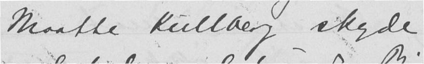Maatte Kullberg skyde
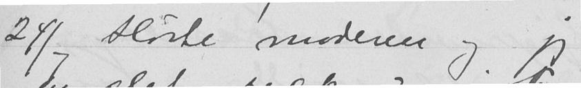24/7 Hørte moderen og jeg
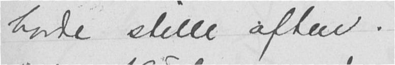havde stille aften.
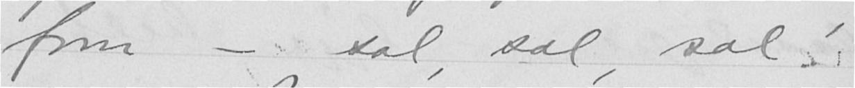form - sol, sol, sol!
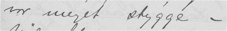var meget stygge -
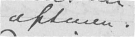aftenen.
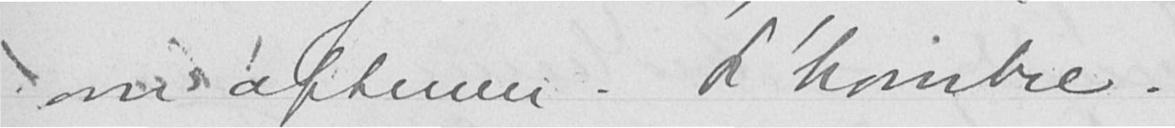om aftenen. L'hombre.
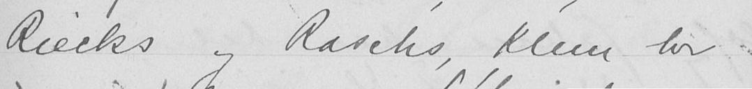Riecks og Raschs, Klem her
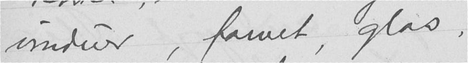vinduer, farvet, glas,
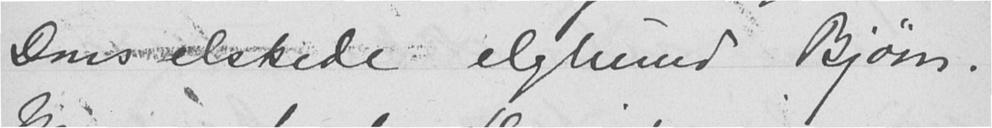Dons elskede elghund Bjørn.
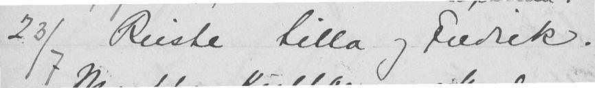23/7 Reiste Lilla og Fredrik.
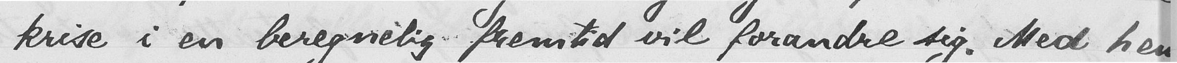krise i en beregnelig fremtid vil forandre sig. Med hensyn
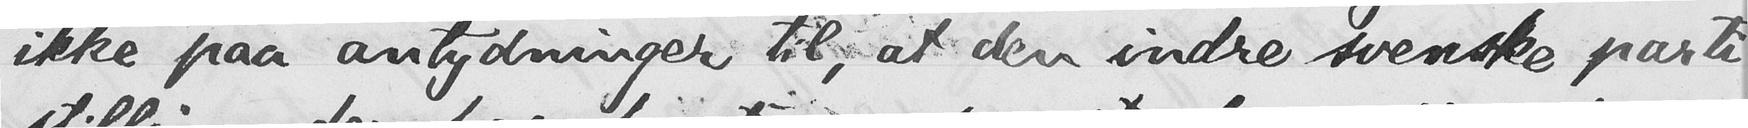ikke paa antydninger til, at den indre svenske parti-
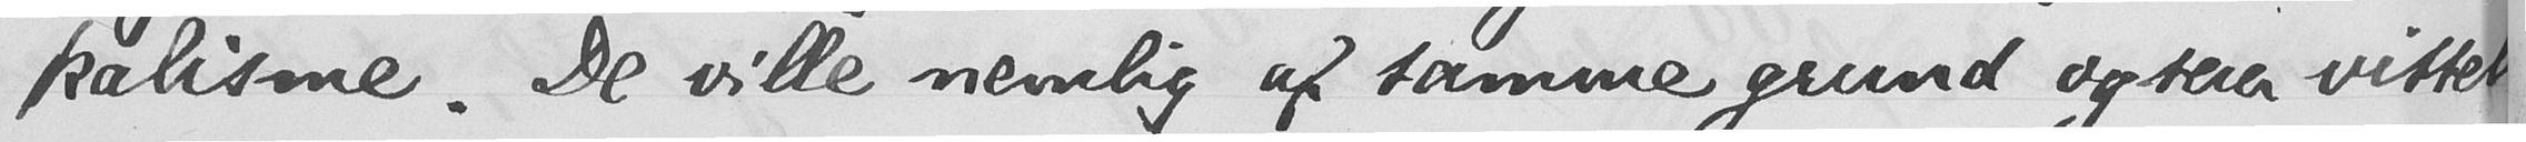kalisme. De ville nemlig af samme grund ogsaa visselig
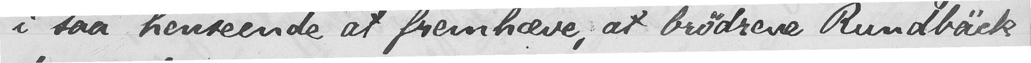i saa henseende at fremhæve, at brødrene Rundbäck og
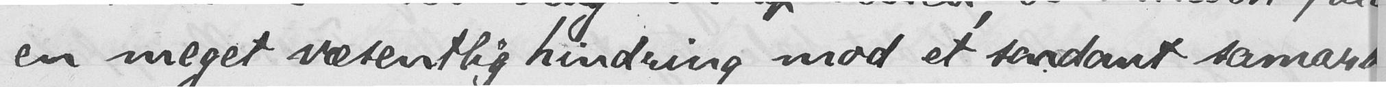en meget væsentlig hindring mod et saadant samarbeid
